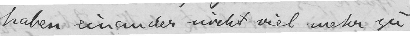haben einander nicht viel mehr zu
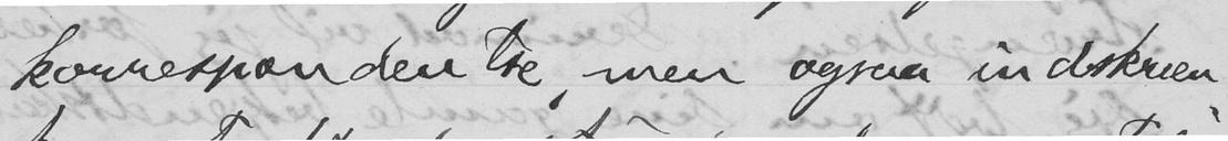korrespondentse, men ogsaa indskræn-
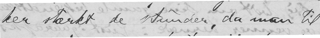ker stærkt de stunder, da man til
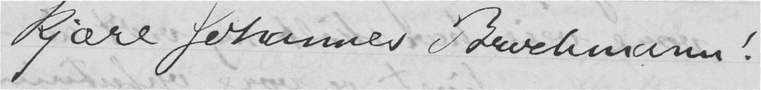Kjære Johannes Brochmann!
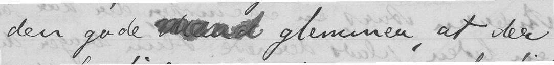den gode  glemmer, at der
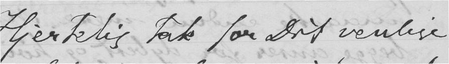Hjertelig tak for Dit venlige
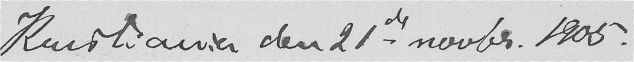Kristiania den 21de novbr. 1905.
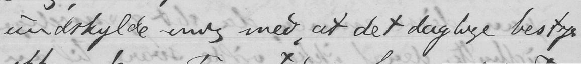undskylde mig med, at det daglige bestyr
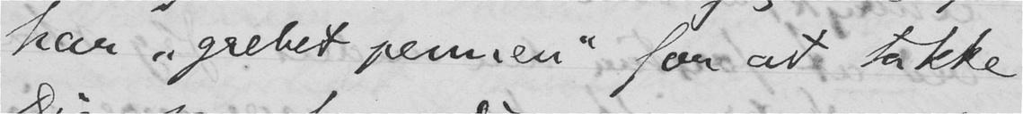har "grebet pennen" for at takke
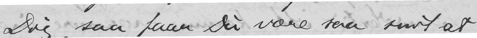Dig, saa faar Du være saa snil at
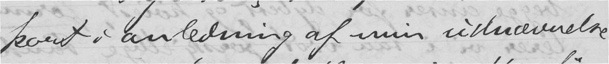kort i anledning af min udnævnelse
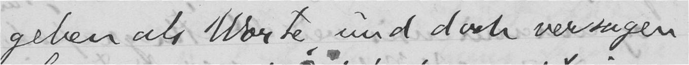geben als Worte, und doch versagen
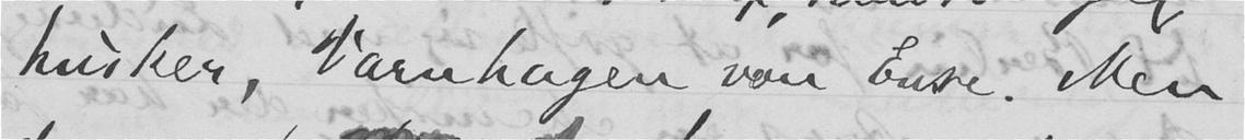husker, Varnhagen von Ense. Men
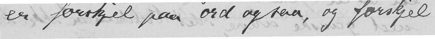er forskjel paa ord ogsaa, og forskjel
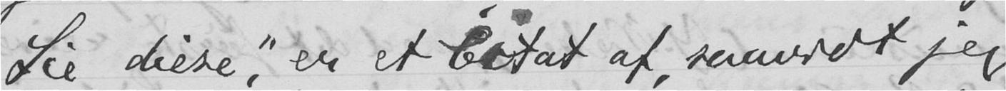Sie diese", er et Citat af, saavidt jeg
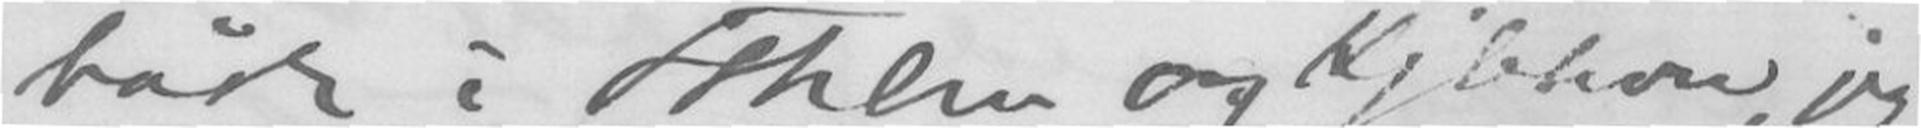både i Sthlm og Kjbhvn, og
